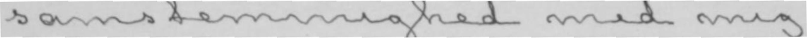samstemmighed med mig.
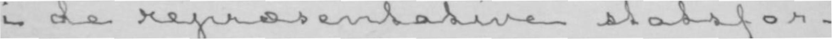i de repræsentative statsfor-
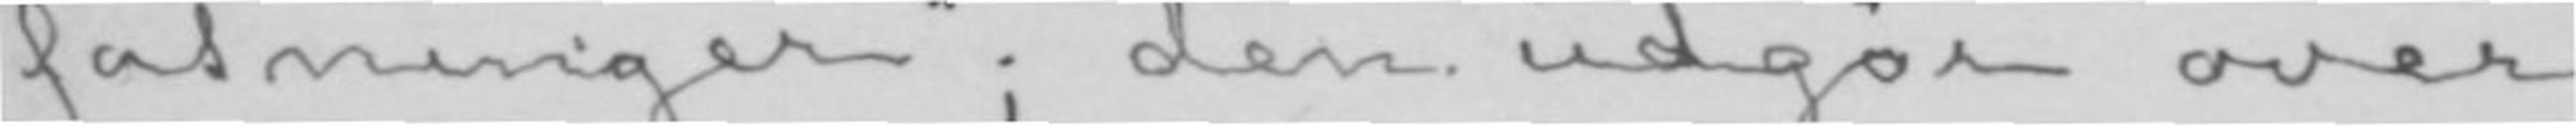fatninger»; den udgør over
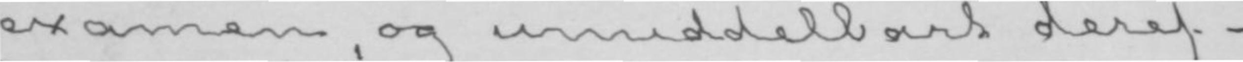examen, og umiddelbart deref-
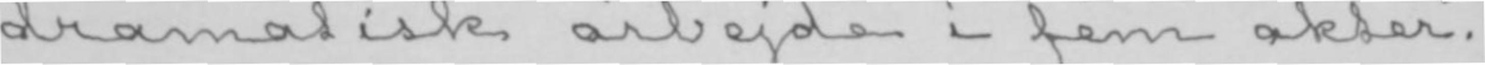dramatisk arbejde i fem akter.
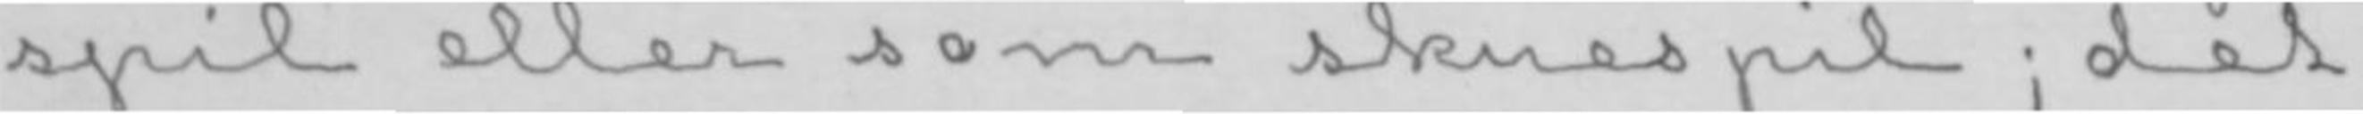spil eller som skuespil; det
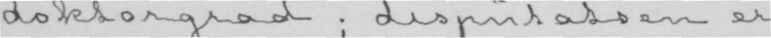doktorgrad; disputatsen er
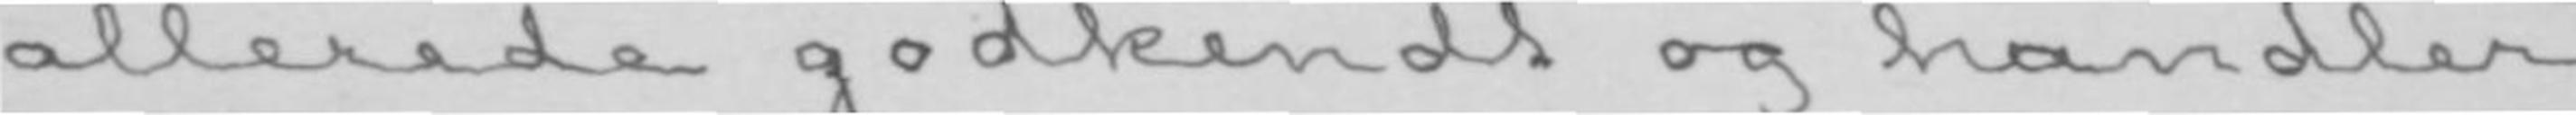allerede godkendt og handler
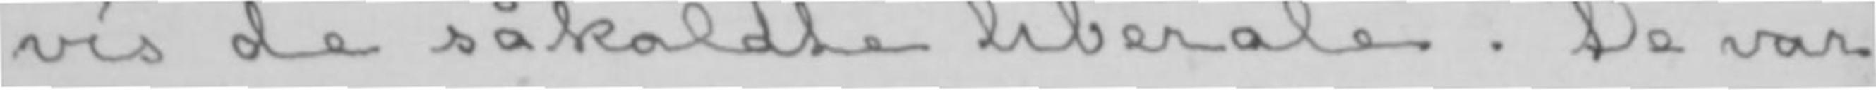vis de såkaldte liberale. De var
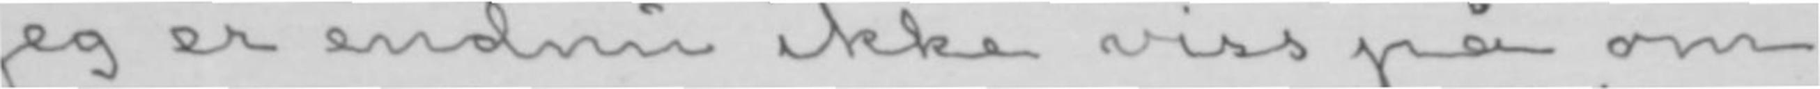Jeg er endnu ikke viss på om
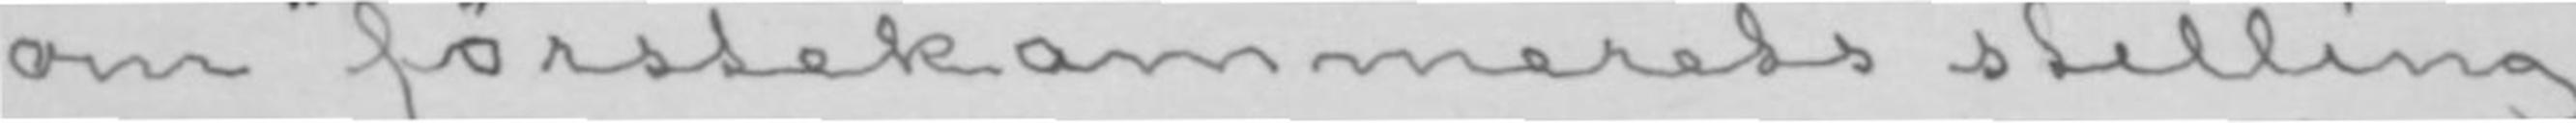om «førstekammerets stilling
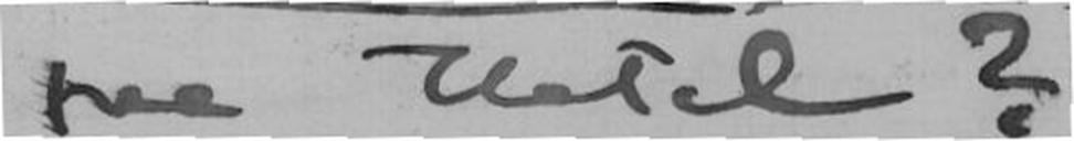paa Hotel ?
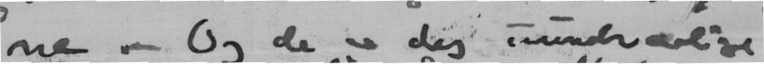ne. Og de er dog uundværlige
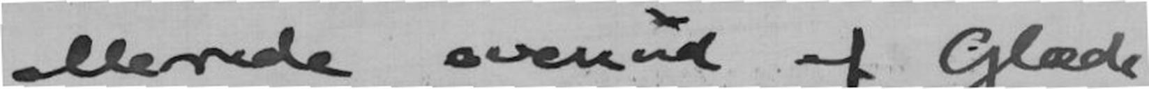allerede ovenud af Glæde
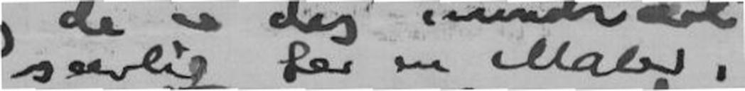særlig for en Maler.
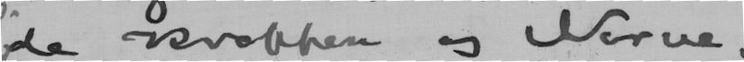da kroppen og Nerve-
